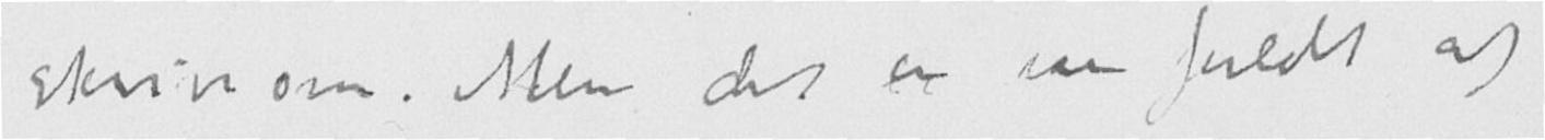skrive om. Men det er saa fuldt af
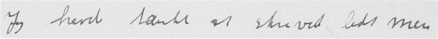Jeg havde tænkt at skrevet lidt mere
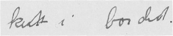ket i bordet.
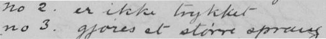no 3. gjøres et større sprang
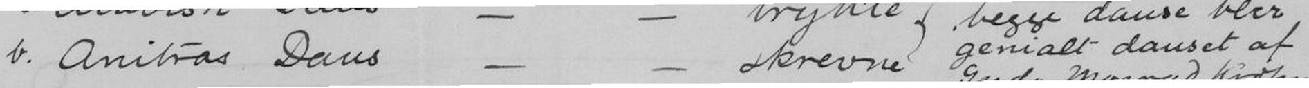skrevne genialt danset af
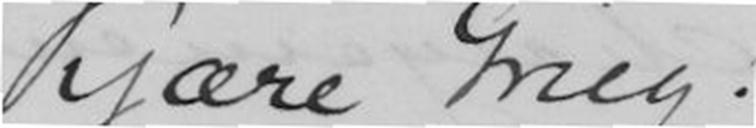Kjære Grieg!
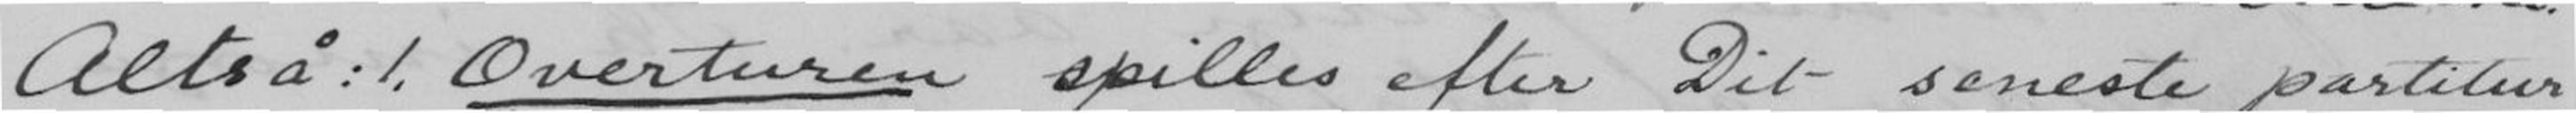Altså: 1. Overturenspilles efter Dit seneste partitur
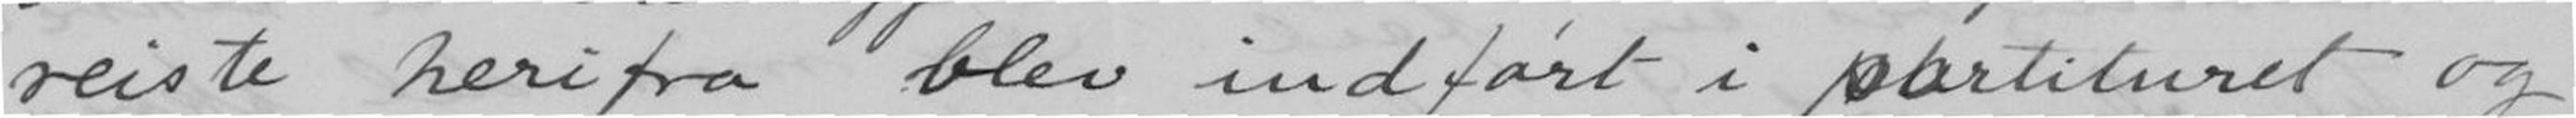reiste herifra blev indført i partituret og
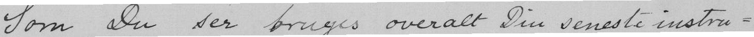Som Du ser bruges overalt Din seneste instru-
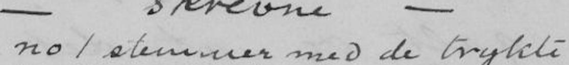no 1 stemmer med de trykte
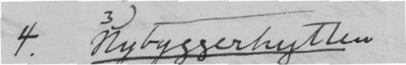4. Nybyggerhytten
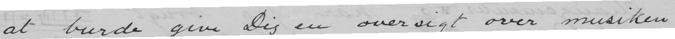at burde give Dig en oversigt over musiken
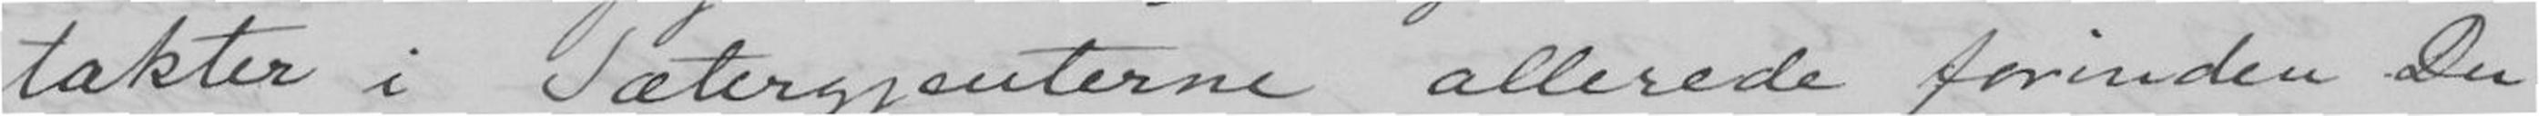takter i Sæterjenterne allerede forinden Du
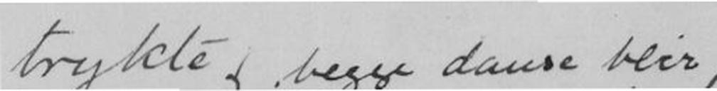trykte {begge danse blir
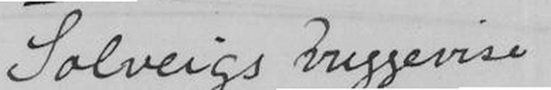Solveigs Vuggevise
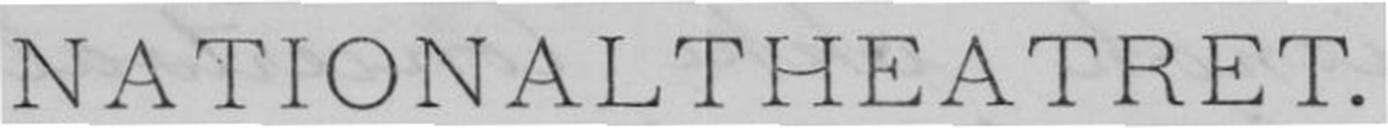NATIONALTHEATRET
